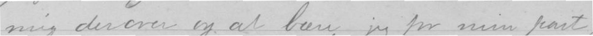mig derover og at bære, jeg for min part,
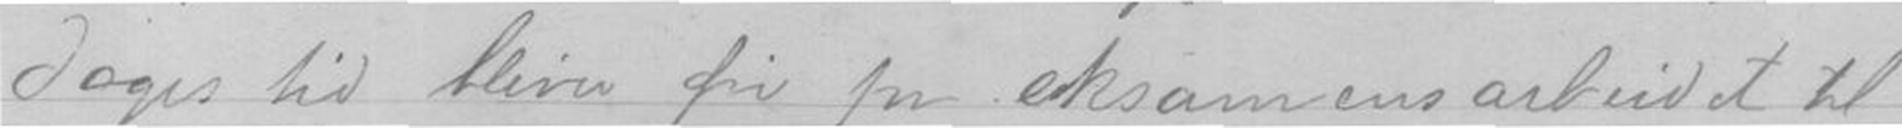Dages tid bliver fri for eksamensarbeidet til
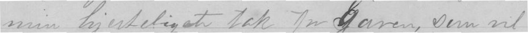min hjerteligste tak for Gaven, som vil
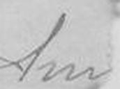An
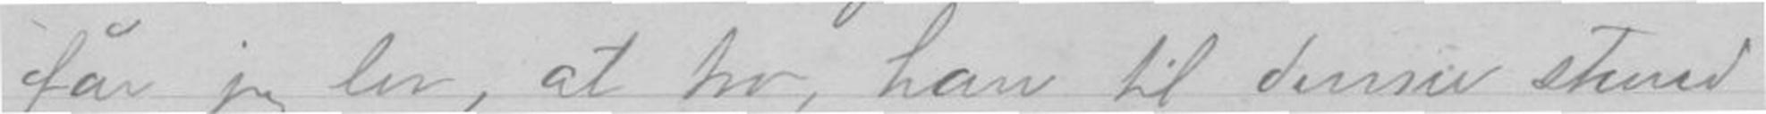får jeg lov, at tro, han til denne stund
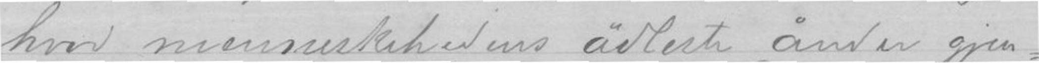hvad menneskehedens ädleste ånder gjen-
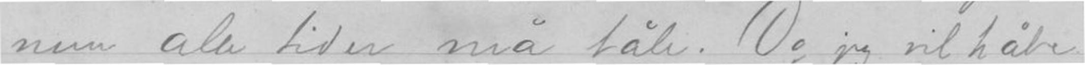nem alle tider må tåle. Og jeg vil håbe
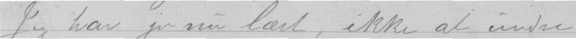Jeg har jo nu lært, ikke at undre
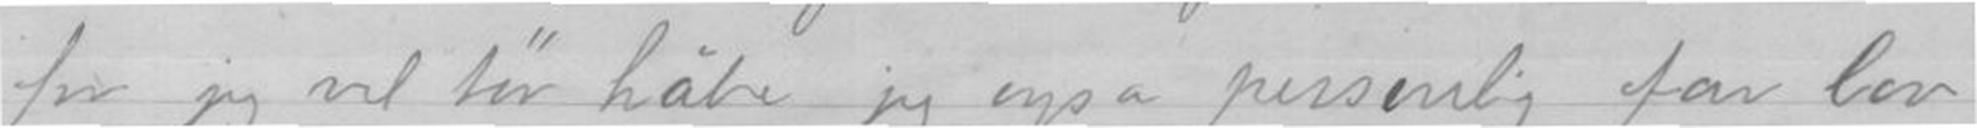for jeg vil tør håbe jeg også personmlig får lov
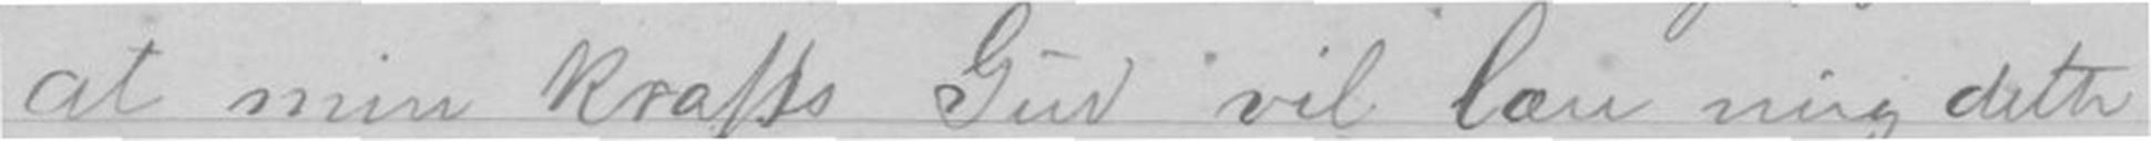at min krafts Gud vil lære mig dette
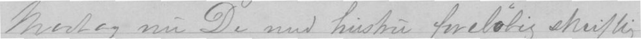Modtag nu De med hustru foreløbig skriftlig
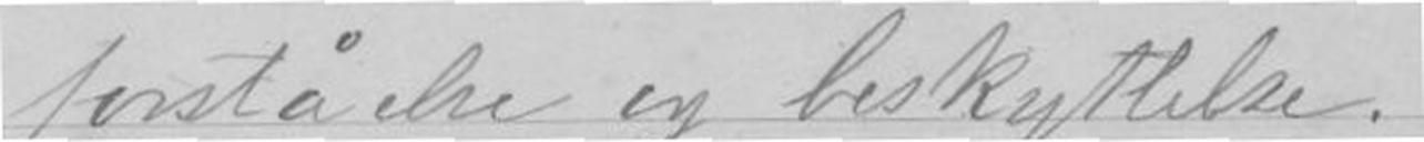forståelse og beskyttelse.
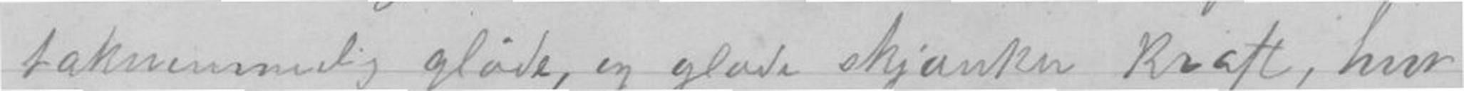taknemmelig gläde, og gläde skjänker kraft, hvor-
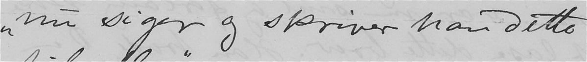"nu siger og skriver han dette
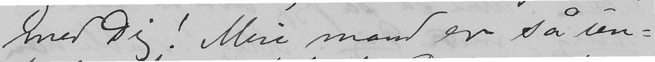med Dig! Min mand er så un-
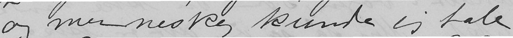og menneske, kunde jeg tale
